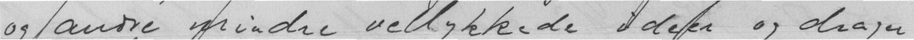og andre mindre vellykkede ideer og drager
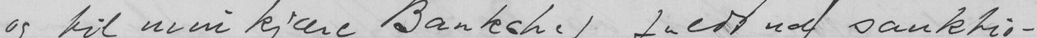og til min kjære Bankchef fuldt ud sanktio-
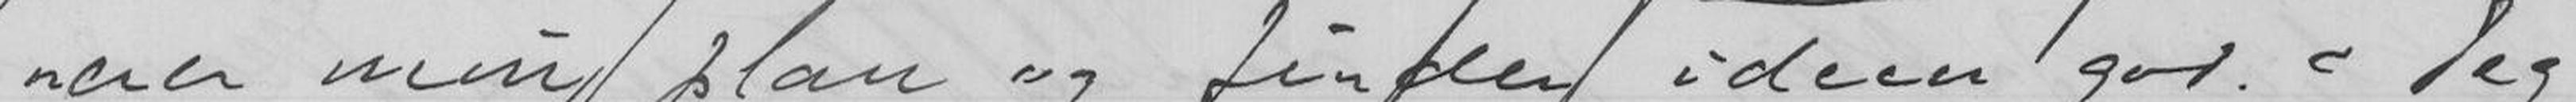nerer min plan og finder ideen god. - Jeg
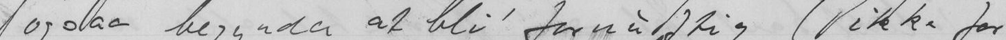ogsaa begynder at bli fornuftig (ikke for
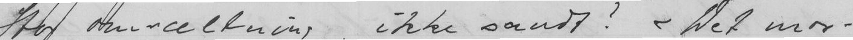Stor omveltning, ikke sandt? Det mor-
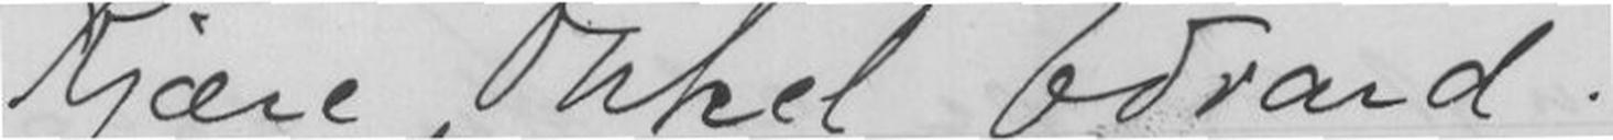Kjære Onkel Edvard!
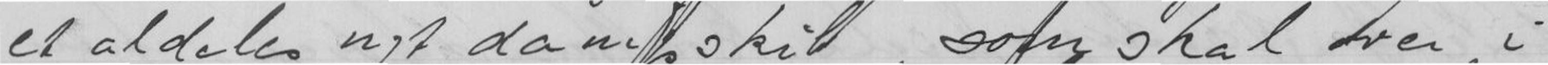et aldeles nyt dampskib, som skal over i
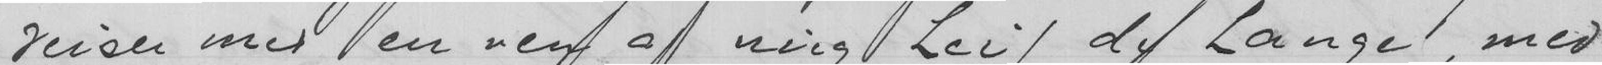reiser med en ven af mig Leif de Lange, med
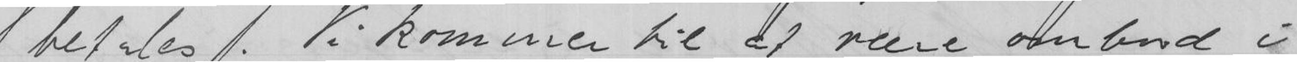betales. Vi kommer til at være ombord i
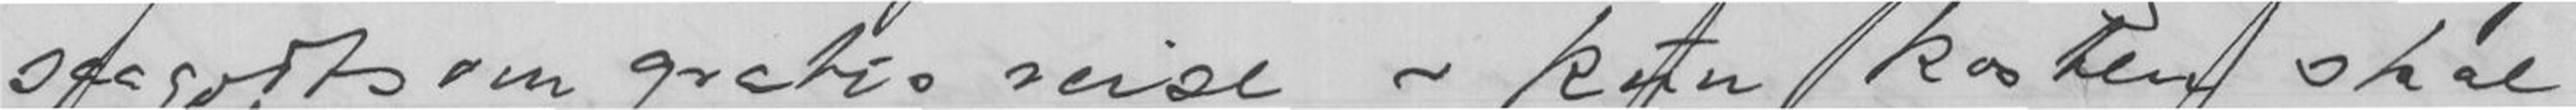saagodtsom gratis reise - kun kosten skal
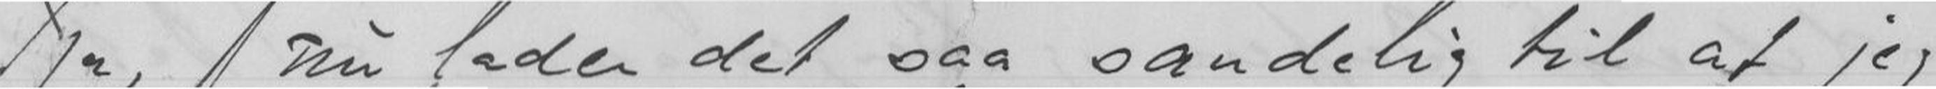Tja, nu lader det saa sandelig til at jeg
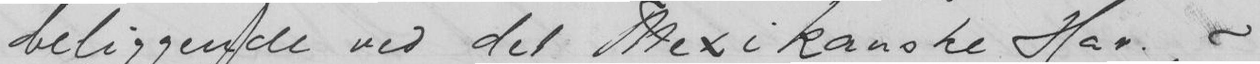beliggende ved det Mexikanske Hav.
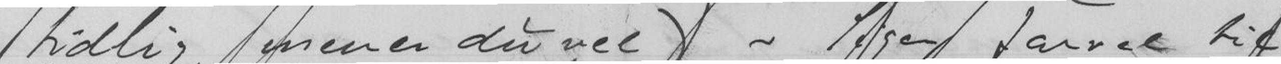tidlig, mener du vel) - Siger farvel til
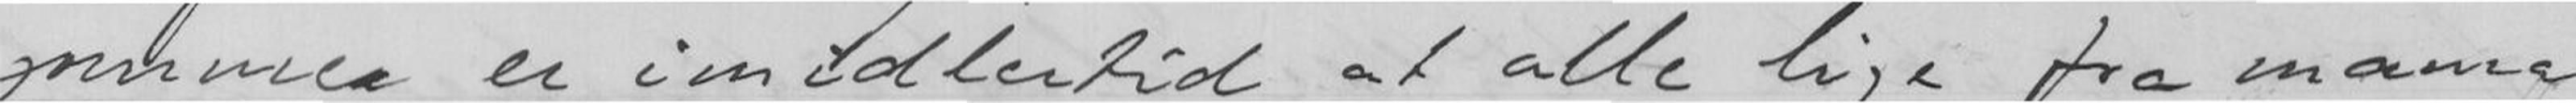somme er imidlertid at alle lige fra mama
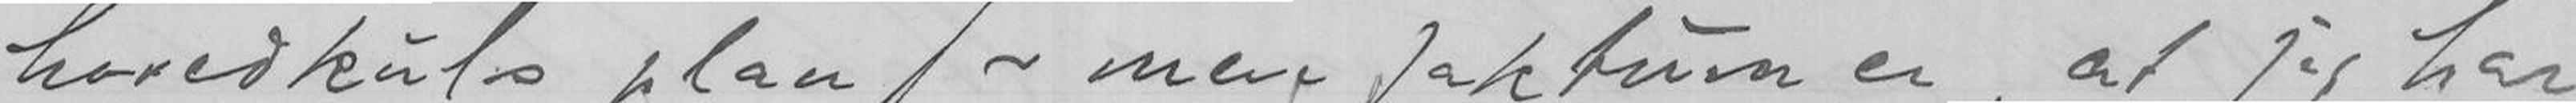hovedkuls plan - men faktum er, at jeg har
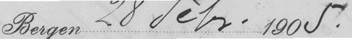Bergen 28 Febr. 1905.
